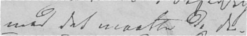med det maatte de der-
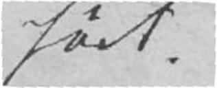ført.
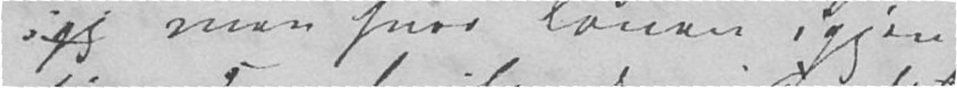men hvor Loven igjen
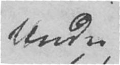Under
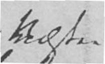Næsten
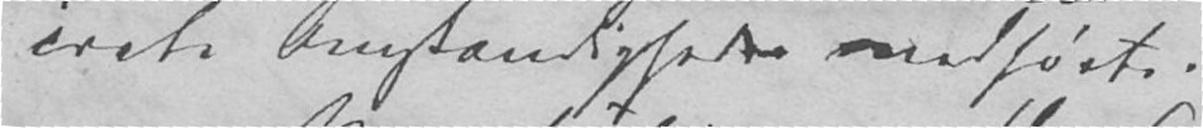crete Omstændigheder medførte.
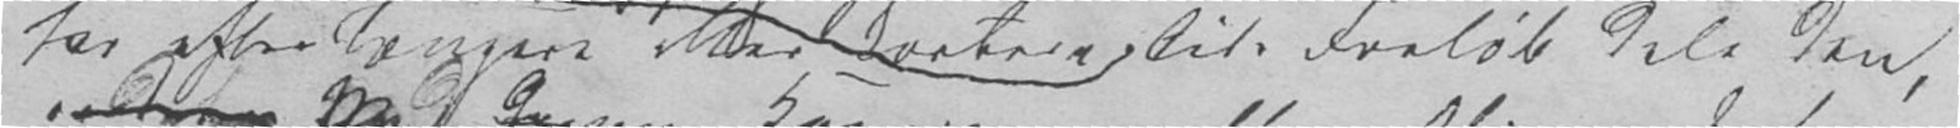for efter længere eller kortere Tids Forløb dele den,
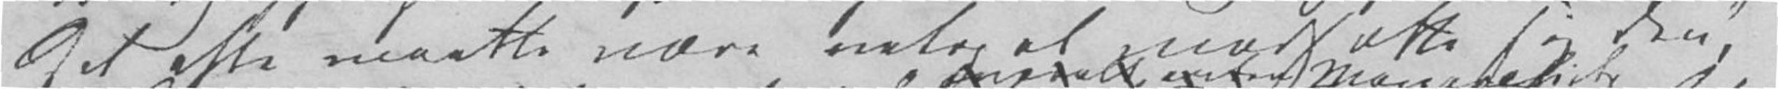det ofte maatte være netop at modsætte sig den,
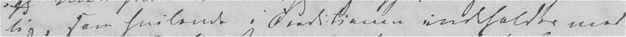lig, som hvilende i Traditionen indeholdes med
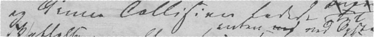og denne Collision ledede til
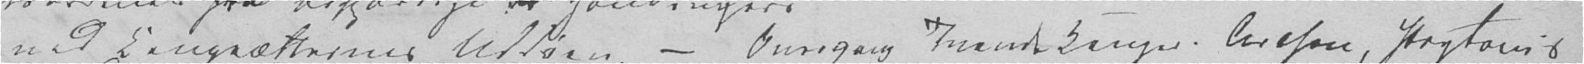ved Kongeætternes Uddøen. – Overgang tvende Konger. Archen, Ptrytonis
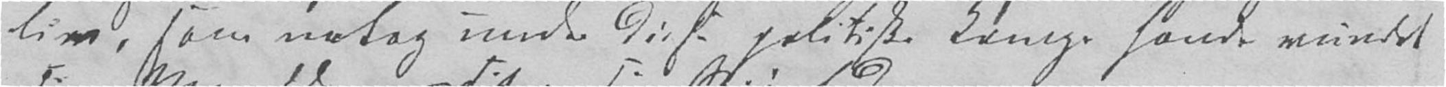liv, som netop under disse politiske Kampe havde vundet
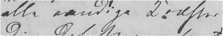alle aandige Kræfter
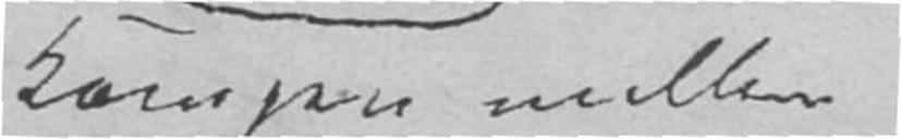Kampen mellem
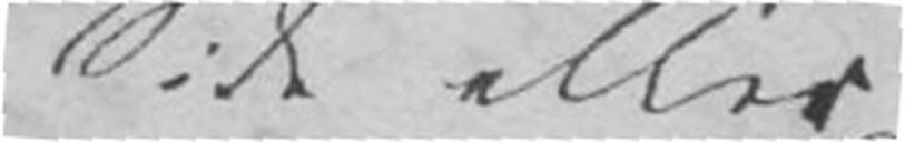Side eller
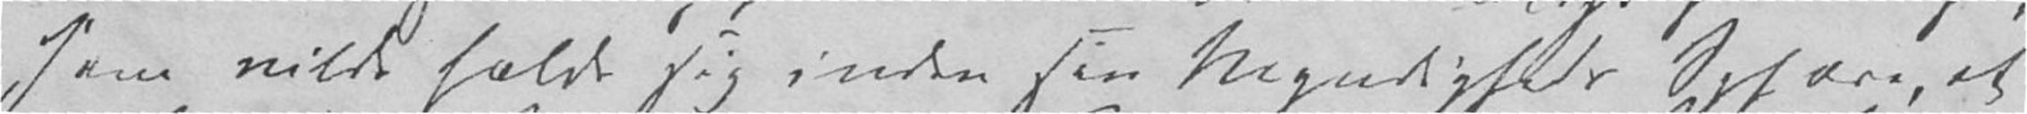som vilde holde sig inden sin Myndigheds Sphære, at
